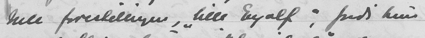hele forestellingen, "lille Eyolf", fordi hun
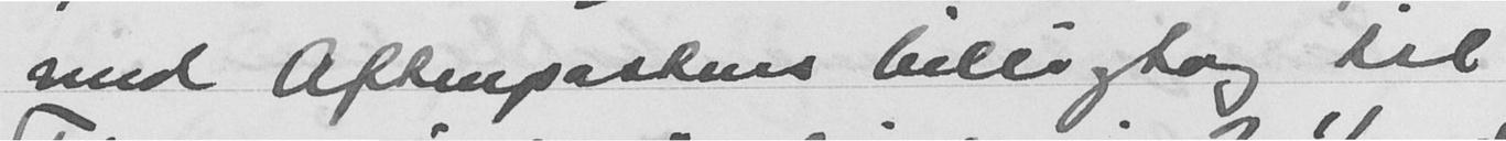med Aftenpostens billigtog til
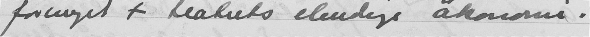formeget + teatrets elendige økonomi.
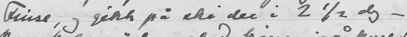Finse, og gikk på ski der i 2 ½ dag -
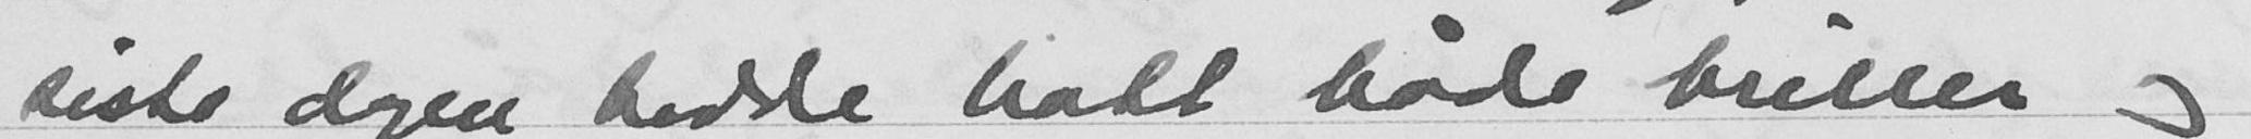siste dagen hadde hatt både briller og
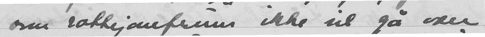som rottejomfruen, ikke vil gå over
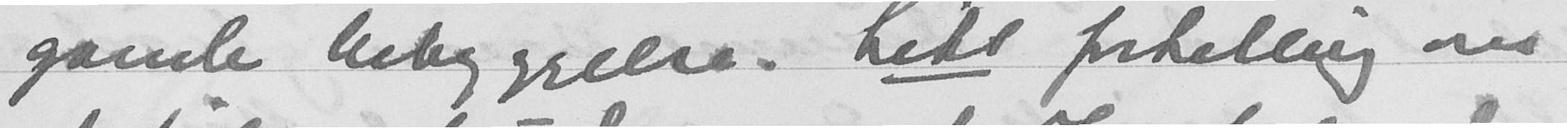gamle bebyggelse. Likt fortelling om
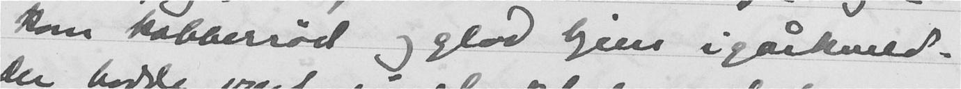kom kobberrød og glad hjem igårkveld-
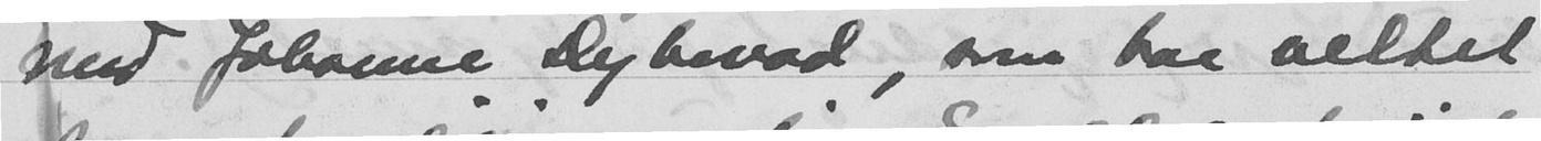med Johanne Dybwad, som har veltet
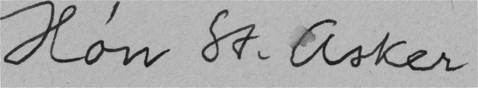Høn St. Asker
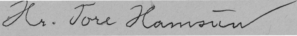Hr. Tore Hamsun
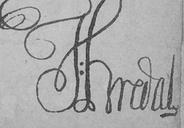T. Bredal
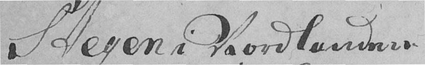Stegen i Nordlandene
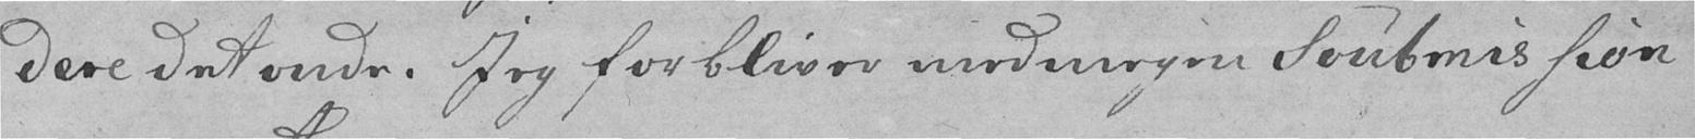dere det onde. Jeg forbliver med megen Soubmission
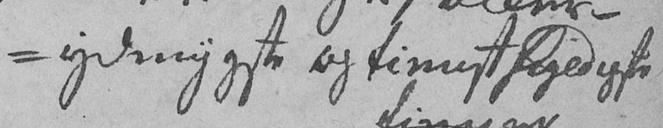ydmygste og tienestskyldigste
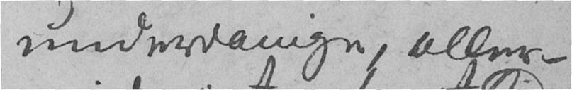underdanige, aller
